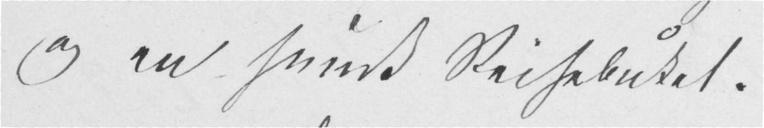og en smuk Reisebuket.
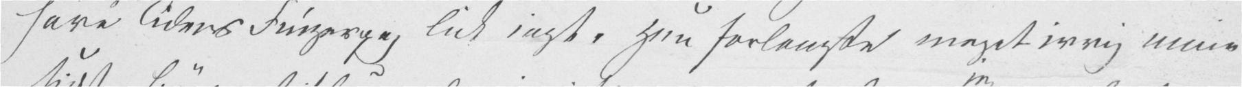have Tidens Fingerpeg lidt ingt. Hun forlangte meget ivrig mine
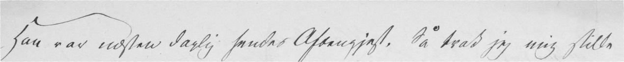Han var næsten daglig hendes Aftengjest. Så trak jeg mig stille
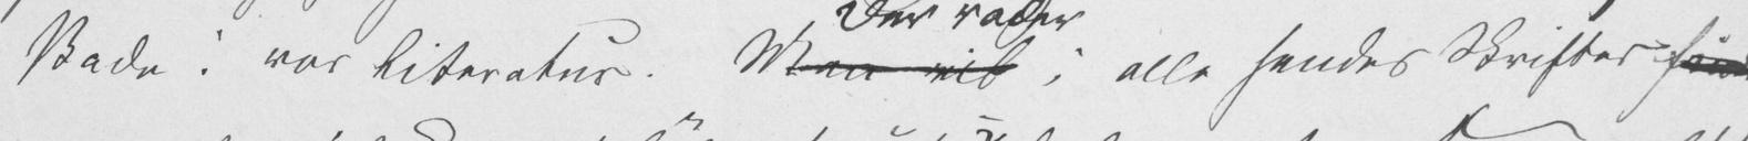skade i vor Literatur. Men vil i alle hendes Skrifter som
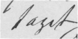taget
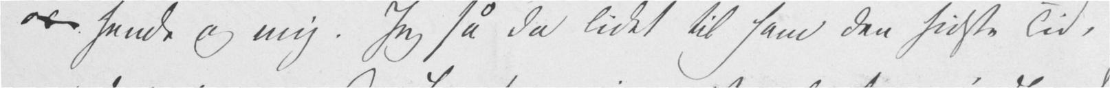os hende og mig. Jeg så da lidet til ham den sidste Tid,
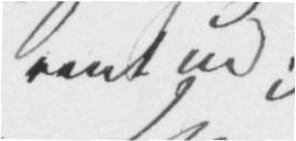rent ud,
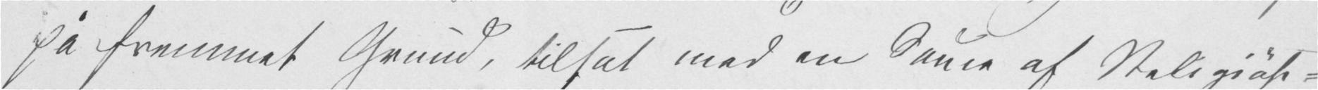på fremmet Grund, tilsat med en Sauce af Religiøsi-
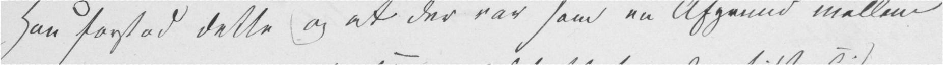Han forstod dette og at der var som en Afgrund mellem
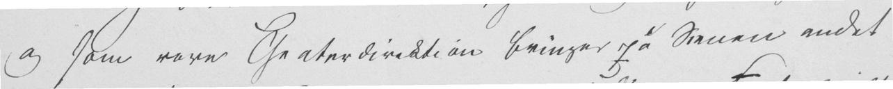og som vore Theaterdirektion bringer på Scenen andet
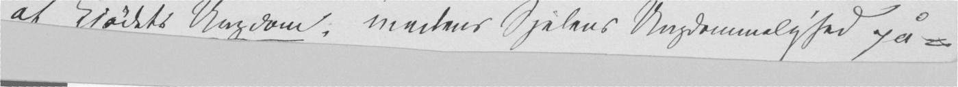af Kjødets Ungdom, medens Sjelens Ungdommelighed på-
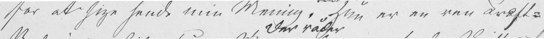for at sige sende min Mening, Hun er en ren Kræft-
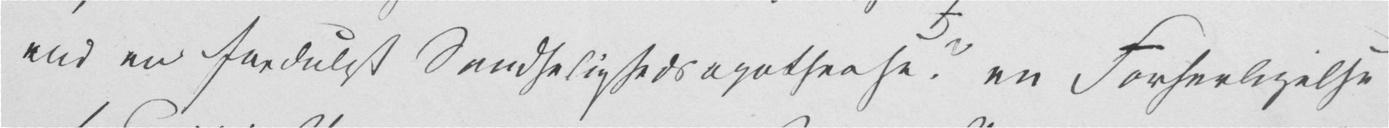end en fordulgt Sandselighedsapothease? en Forherligelse
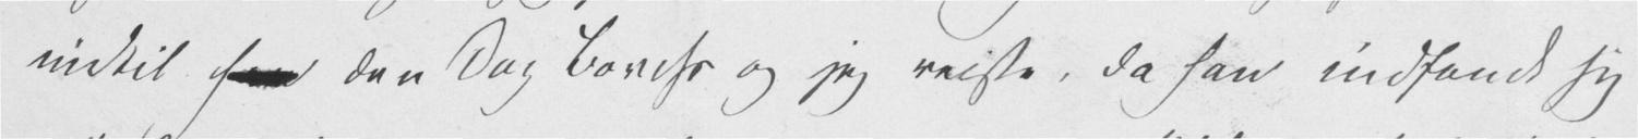indtil han den Dag Borchs og jeg reiste, da han indfandt sig
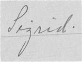Sigrid.
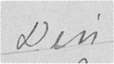Din
